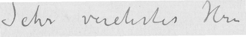Sehr verehrter Hrn
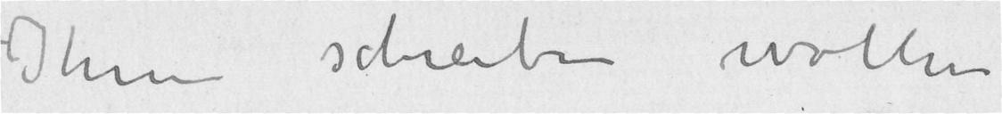Ihnen schreiben wollen
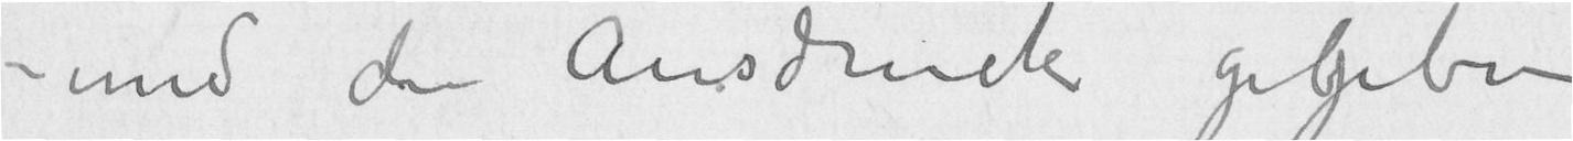– und den Ausdruck gegeben
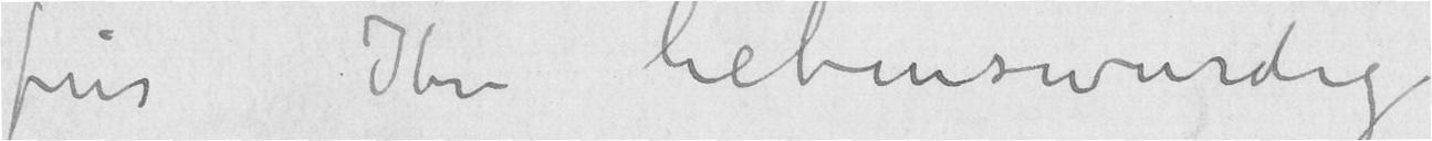für Ihre liebenswurdig
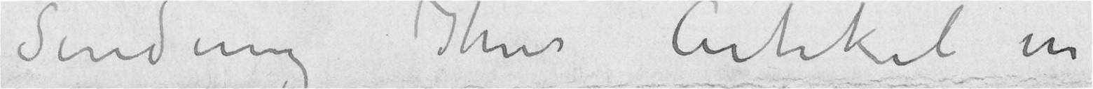Sendung Ihres Artikel in
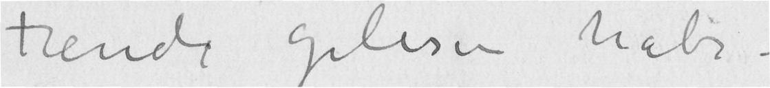Freude gelesen habe –
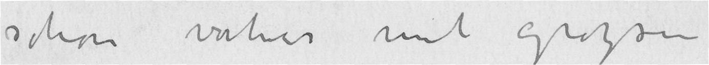schon vorher mit groszer
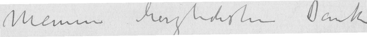Meinen herzlichsten Dank
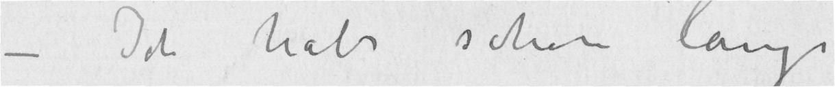– Ich habe schon lange
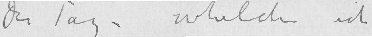Der Tag – whelche ich
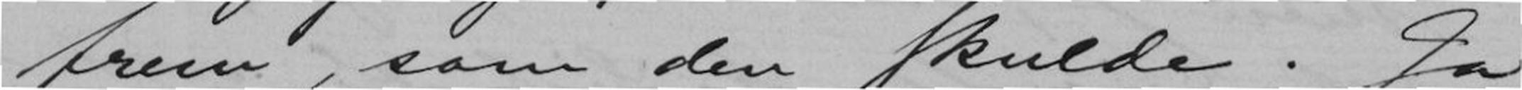frem, som den skulde. Ja,
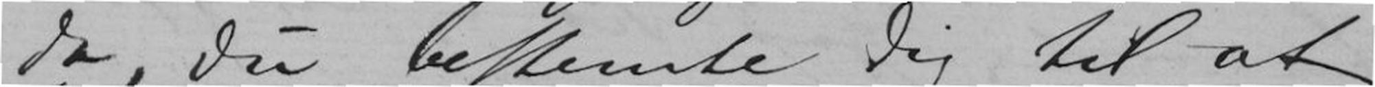da, du bestemte dig til at
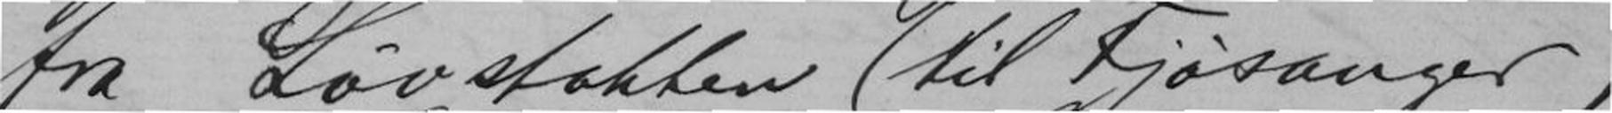fra Løvstakken (til Fjøsanger)
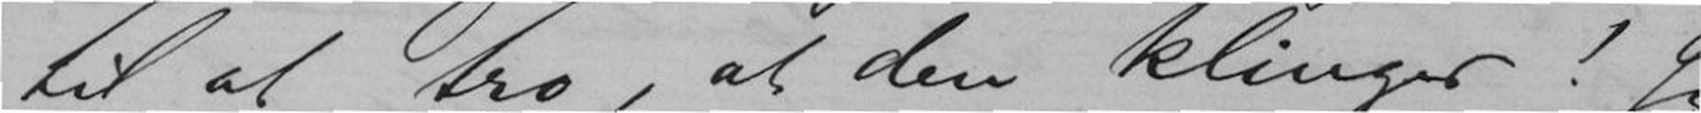til at tro, at den klinger! Jeg
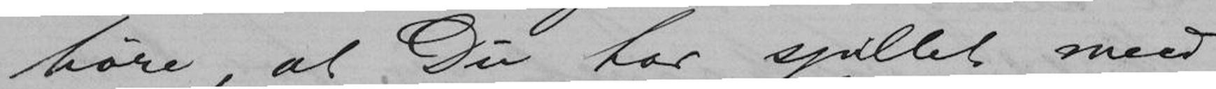høre, at Du har spillet med
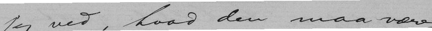jeg ved, hvad den maa være,
